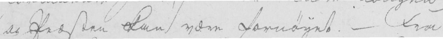og Præsten kan være fornøyet. - Fra
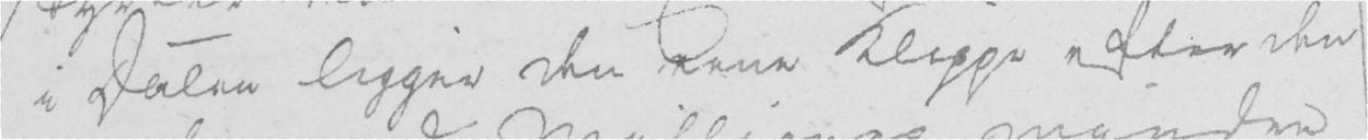i Dalen ligger den eene klippe efter den
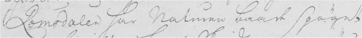Romsdalen har Naturen baade spøget
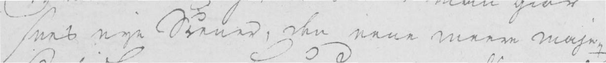sees nye Scener, den eene meere maje-
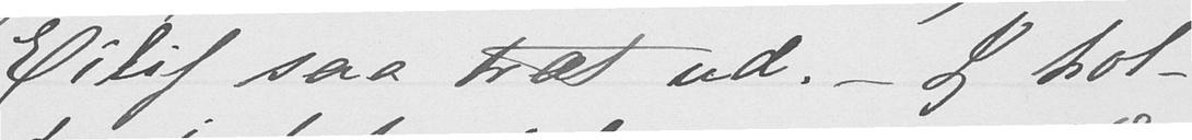Eilif saa træt ud. - Jeg hol-
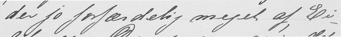der jo forfærdelig meget af Ei-
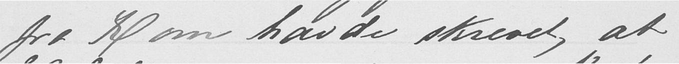fra Rom havde skrevet, at
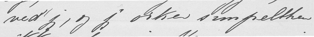ved jeg, og jeg orker simpelthen
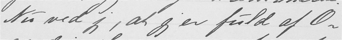Nu ved jeg, at jeg er fuld af O-
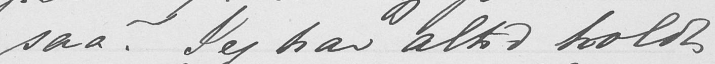saa. Jeg har altid holdt
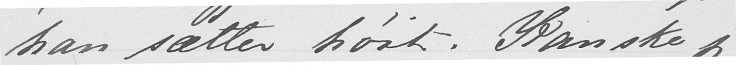han sætter høit. Kanske jeg
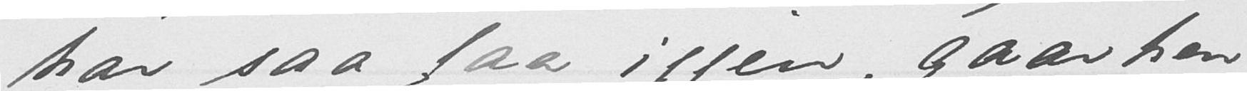har saa faa igjen, gaar hen
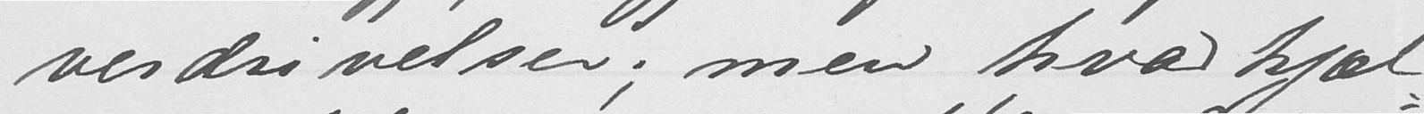verdrivelser; men hvad hjæl-
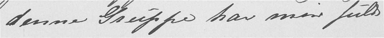denne Gruppe har min fulde
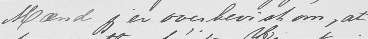Mænd jeg er overbevist om, at
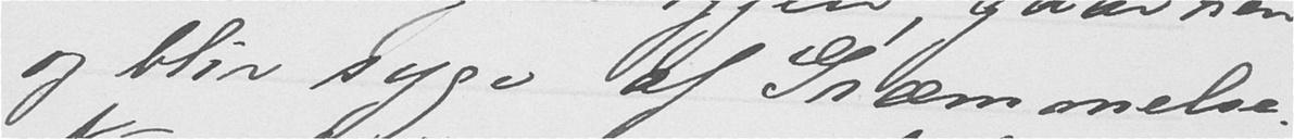og blir syge af Græmmelse.
